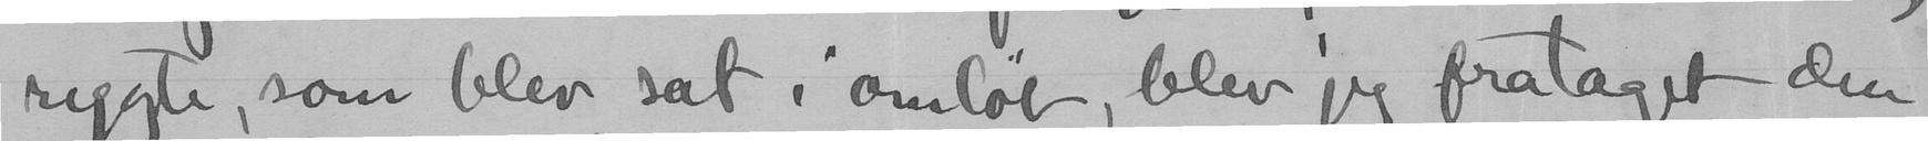rygte, som blev sat i omløb, blev jeg frataget den
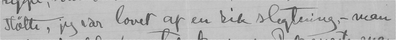støtte, jeg var lovet af en rik slegtning, - man
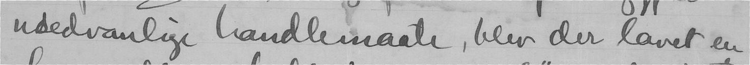usedvanlige handlemaate, blev der lavet en
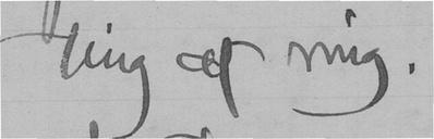ting af mig.
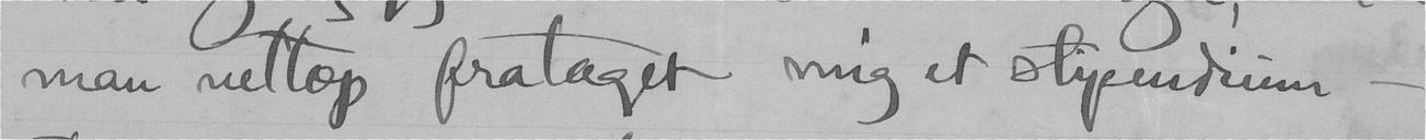man nettop frataget mig et stipendium -
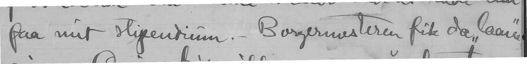faa mit stipendium. - Borgermasteren fik da "laane"
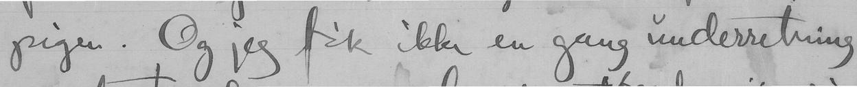pigen. Og jeg fik ikke en gang underretning
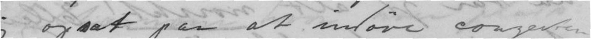jeg opsat paa at indøve conzerten
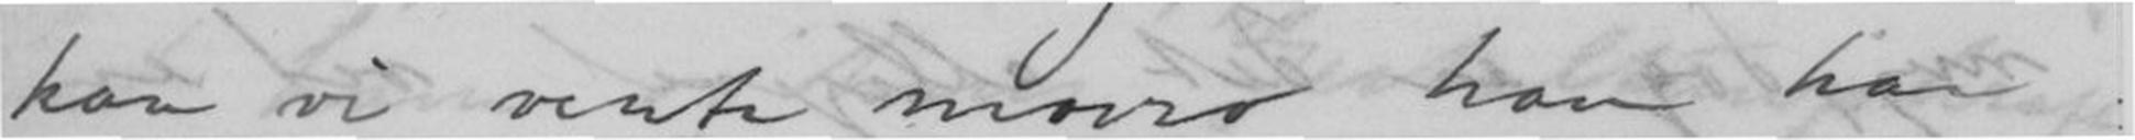kan vi vente morro, han har
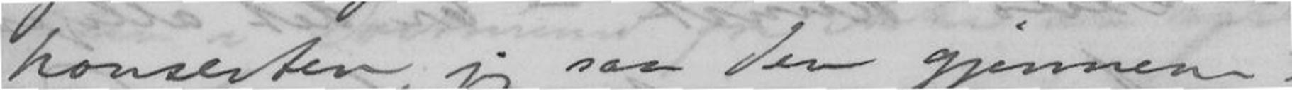konserten, jeg saa den gjennem
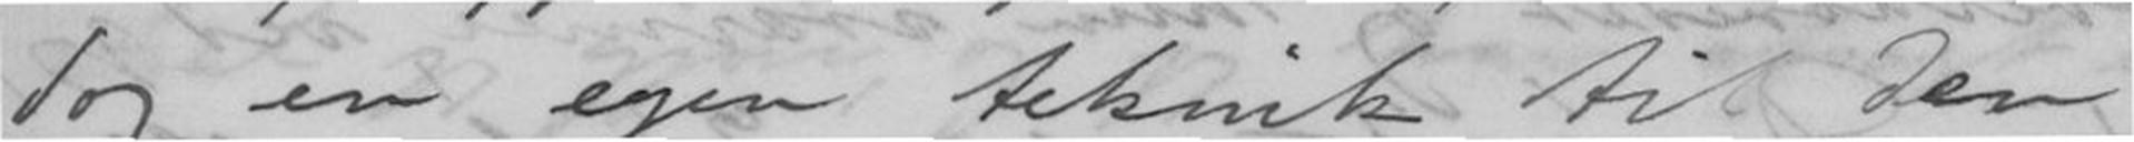dog en egen teknik til den
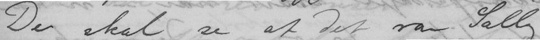De skal se at det var Sally
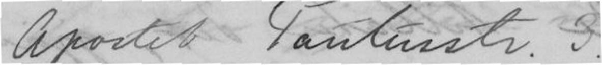Apostel Paulusstrasse 3
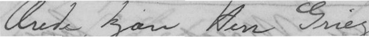Ærede kjære Herr Grieg!
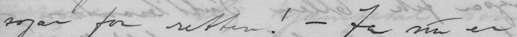sogar for retten! - Ja nu er
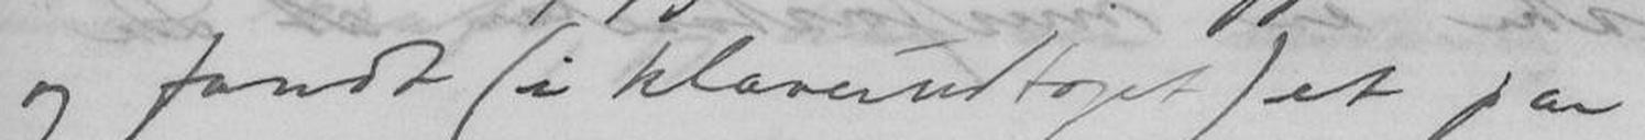og fandt (i klaverudtoget) et par
